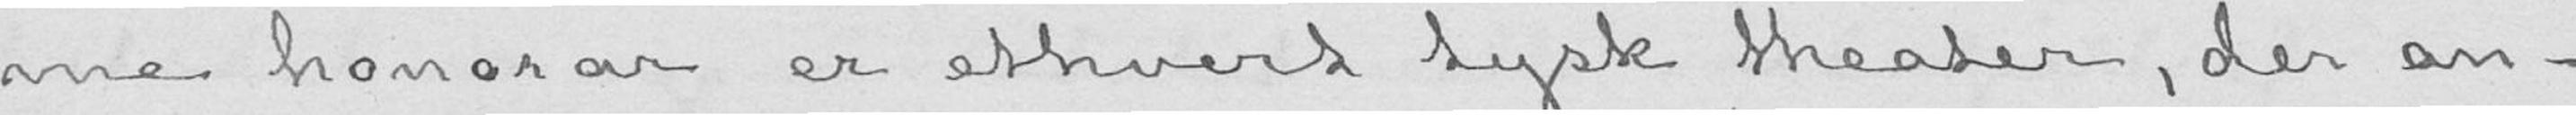me honorar er ethvert tysk theater, der an-
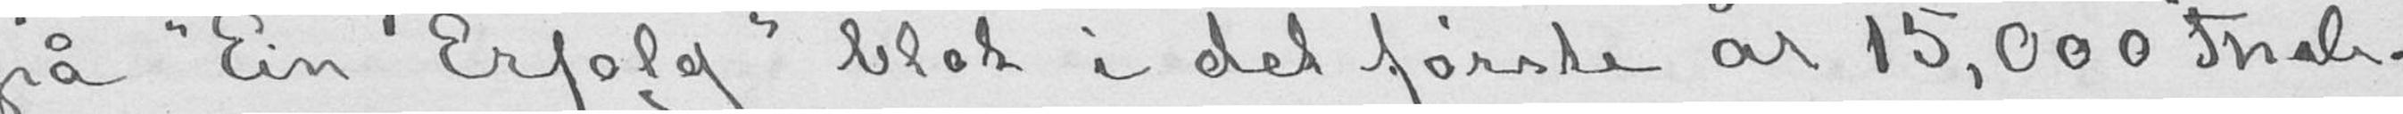på «Ein Erfolg» blot i det første år 15,000 Thaler.
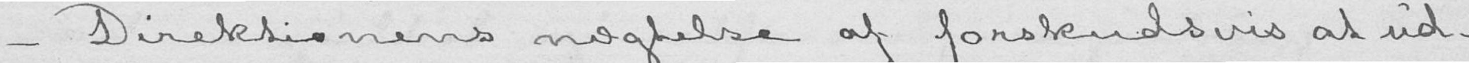- Direktionens nægtelse af forskudsvis at ud-
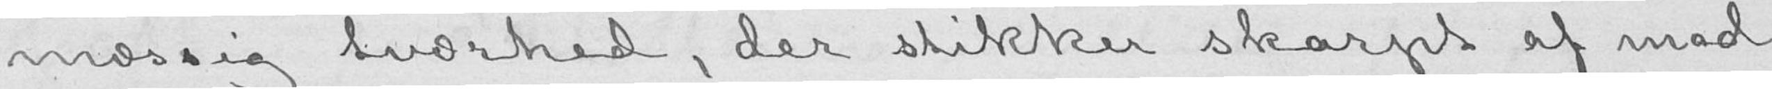mæssig tværhed, der stikker skarpt af mod
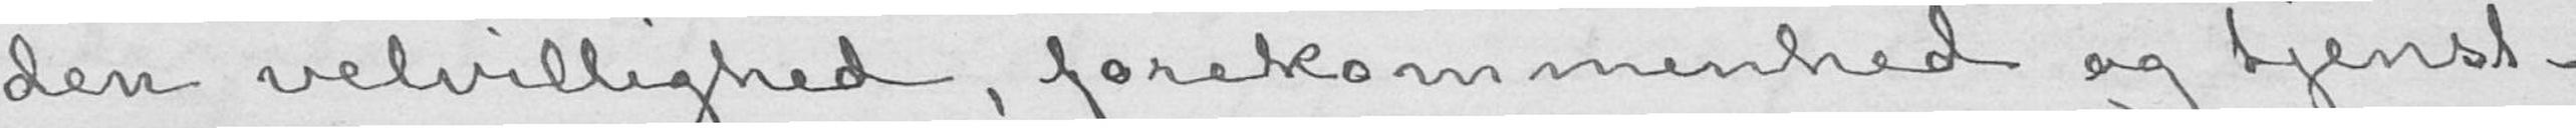den velvillighed, forekommenhed og tjenst-
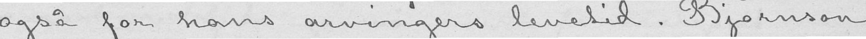også for hans arvingers levetid. Bjørnson
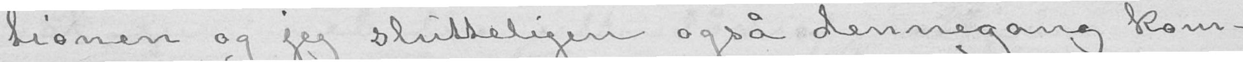tionen og jeg slutteligen også dennegang kom-
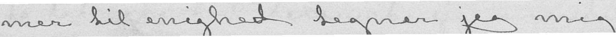mer til enighed tegner jeg mig
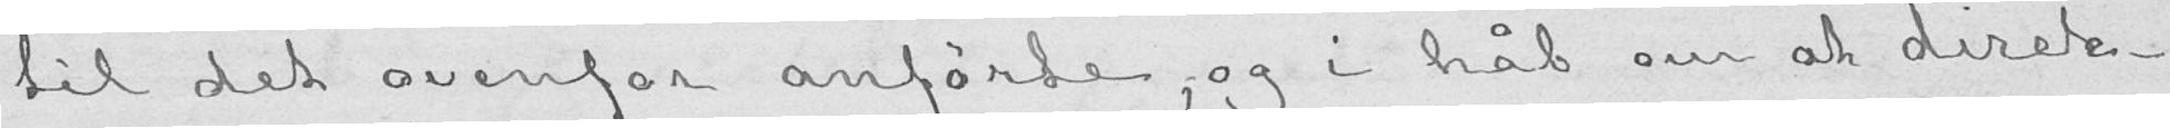til det ovenfor anførte, og i håb om at direk-
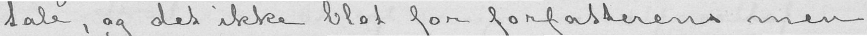tale, og det ikke blot for forfatterens men
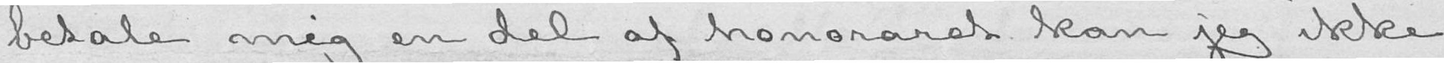betale mig en del af honoraret kan jeg ikke
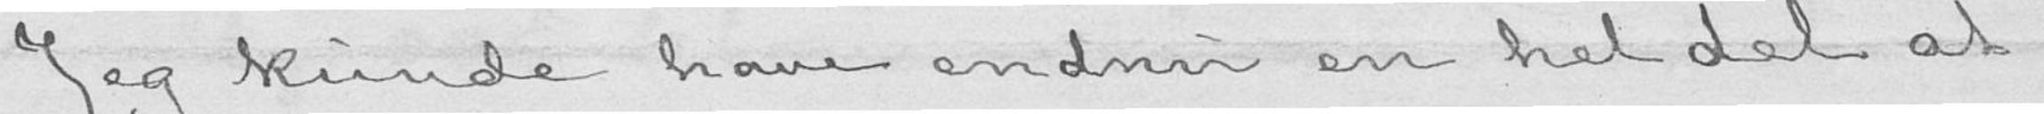Jeg kunde have endnu en hel del at
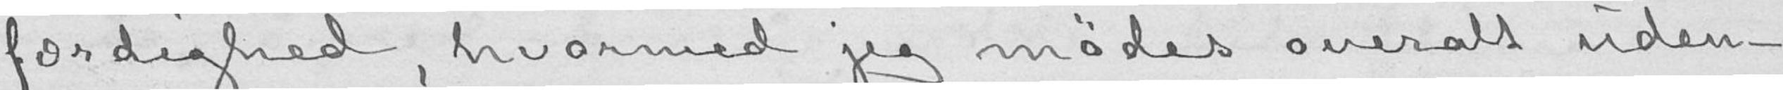færdighed, hvormed jeg mødes overalt uden-
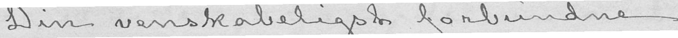Din venskabeligst forbundne
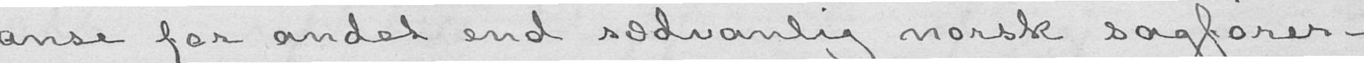anse for andet end sædvanlig norsk sagfører-
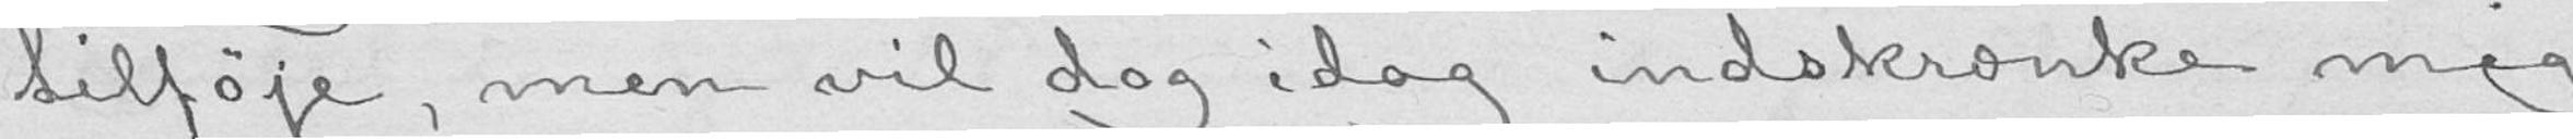tilføje, men vil dog idag indskrænke mig
